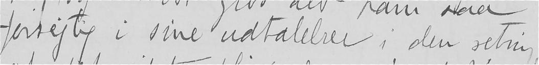forsigtig i sine udtalelser i den retning,
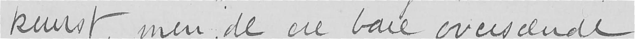kunst, men de ere bare overseende
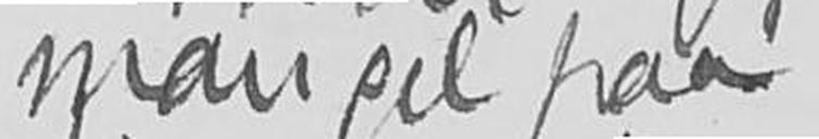mangel paa
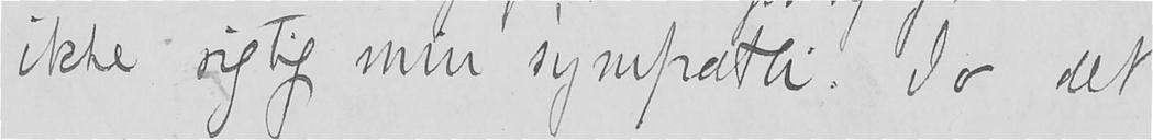ikke rigtig min sympathi. Jo det
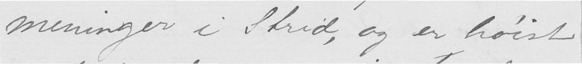meninger i Strid, og er høist
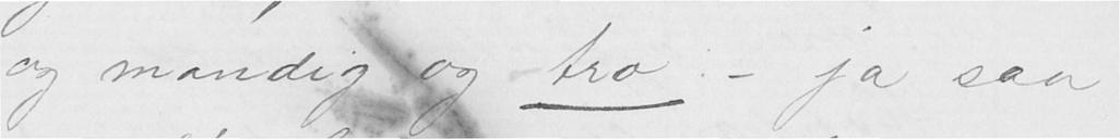og mandig og tro — ja saa
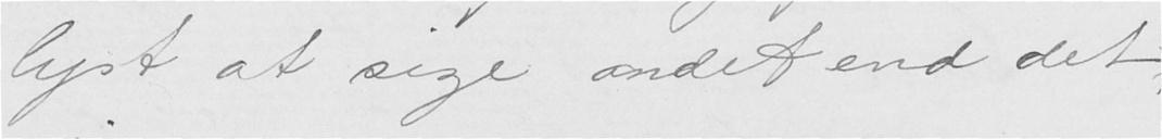lyst at sige andet end det,
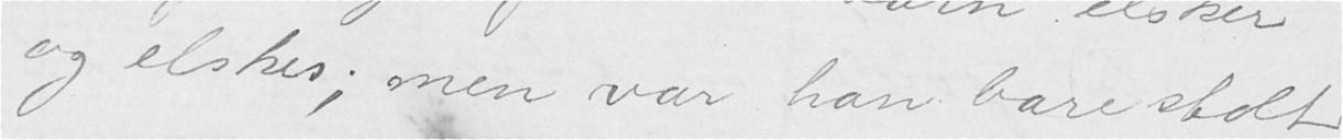og elskes; men var han bare stolt
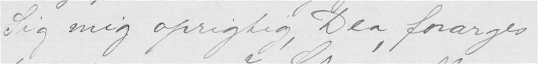Sig mig oprigtig, Dea, forarges
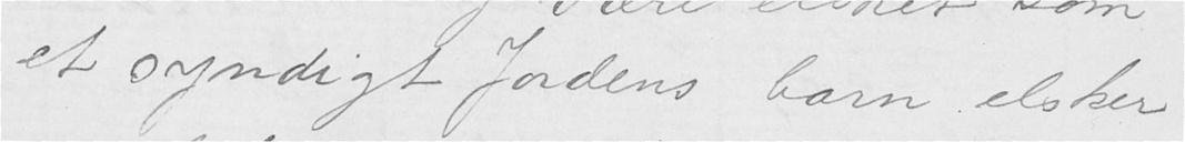et syndigt Jordens barn elsker
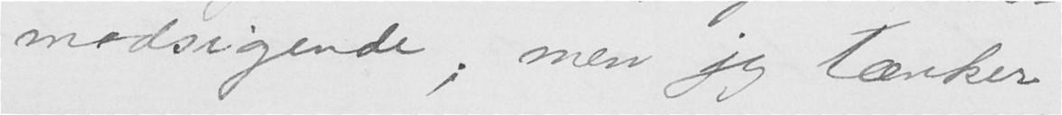modsigende; men jeg tænker
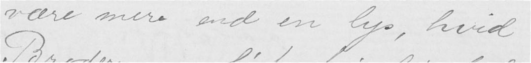være mere end en lys, hvid
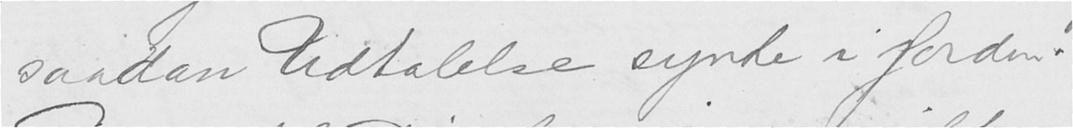saadan Udtalelse synke i Jorden!
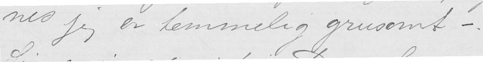nes jeg er temmelig grusomt —.
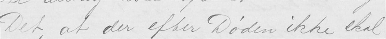Det, at der efter Døden ikke skal
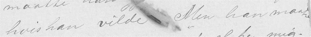hvis han vilde. Men han maat-
